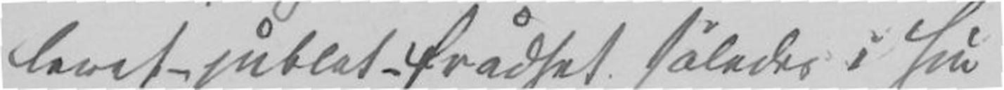levet-jublet-frådset således i sin
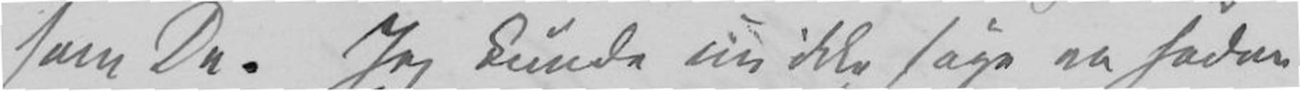som De. Jeg kunde nu ikke søge en sådan
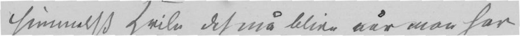himmelsk Hvile det må blive når man har
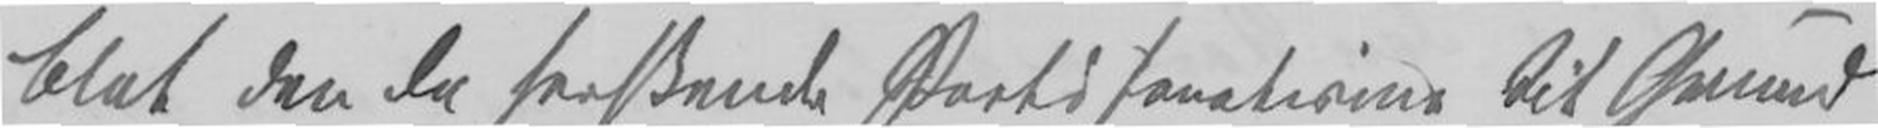blot den da forskunde Parti fanatisme til Grund
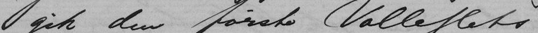gik den første Valleslets-
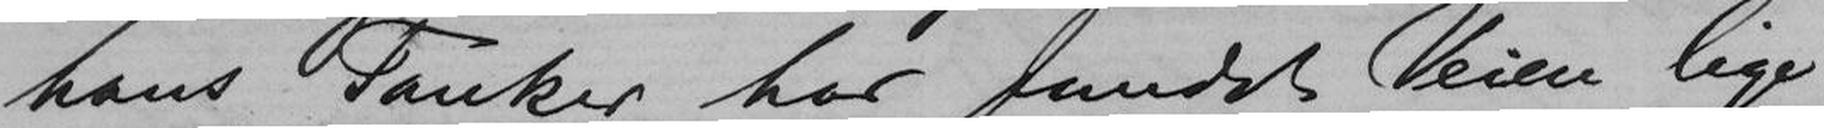hans Tanker har fundet Veien lige
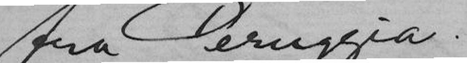fra Peruggia.
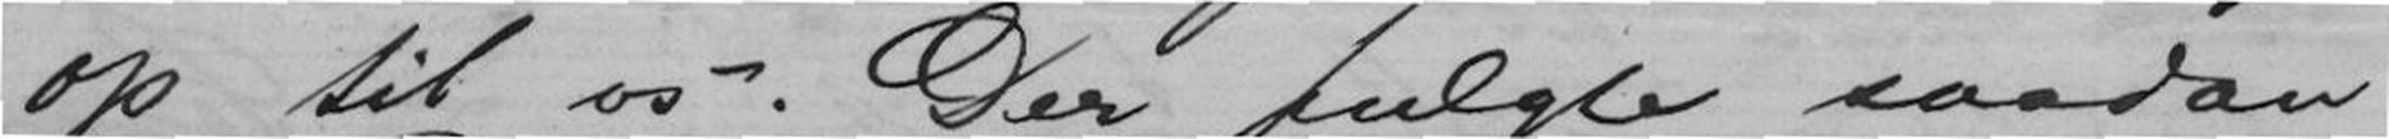op til os. Der fulgte saadan
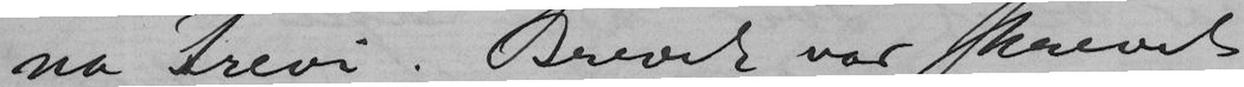na Trevi. Brevet var skrevet
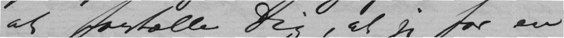at fortælle Dig, at jeg for en
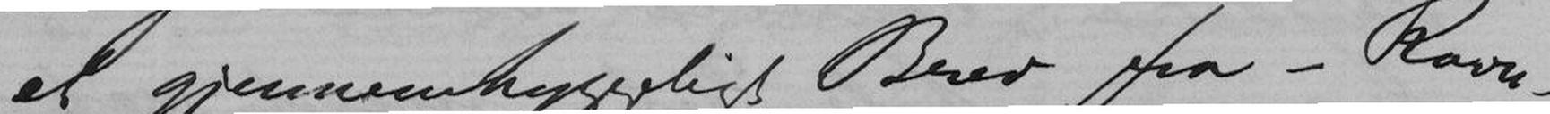et gjennemhyggeligt Brev fra - Ravn-
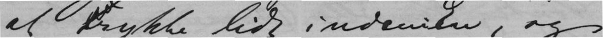at rykke lidt indeni En, og
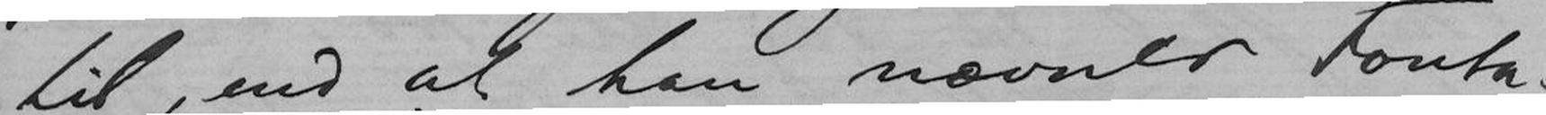til, end at han nævner Fonta-
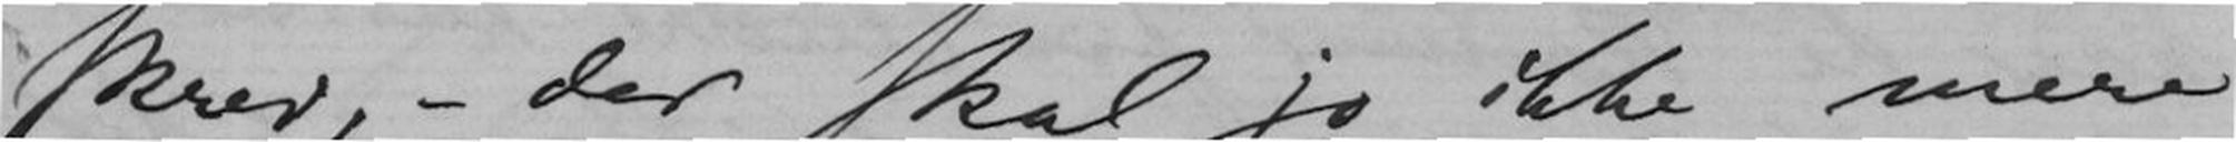skrev, - der skal jo ikke mere
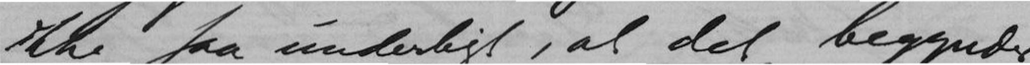ikke saa underligt, at det begynder
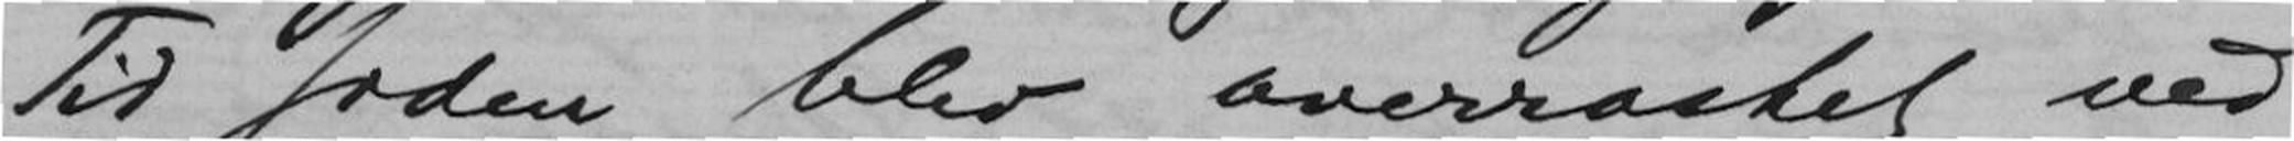Tid siden blev overrasket ved
